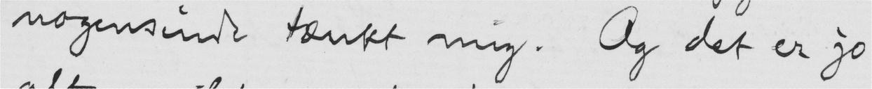nogensinde tænkt mig. Og det er jo
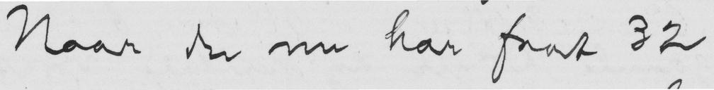Naar du nu har faat 32
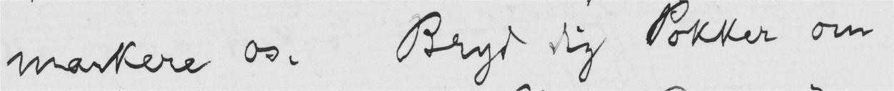markere os. Bryd dig Pokker om
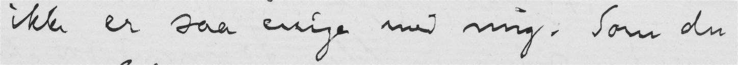ikke er saa enige med mig. Som du
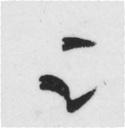i
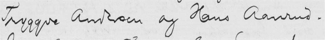Tryggve Andersen og Hans Aanrud.
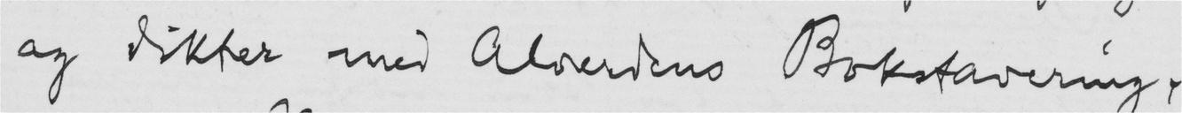og dikter med Alverdens Bokstavering,
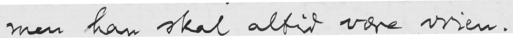men han skal altid være vrien.
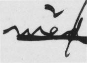med
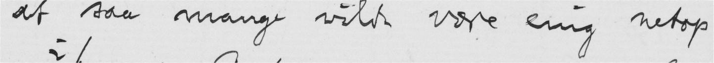at saa mange vilde være enig netop
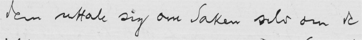dem uttale sig om Saken selv om de
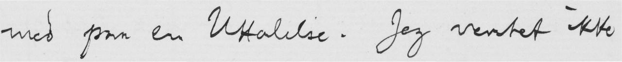med paa en Uttalelse. Jeg ventet ikke
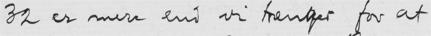32 er mere end vi trænger for at
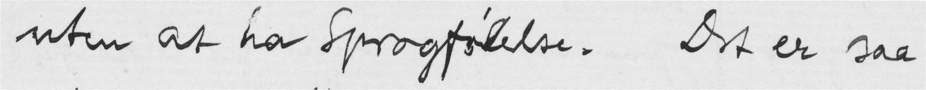uten at ha Sprogfølelse. Det er saa
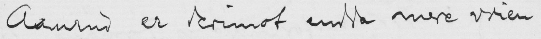Aanrud er derimot endda mere vrien
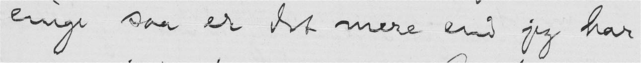enige saa er det mere end jeg har
